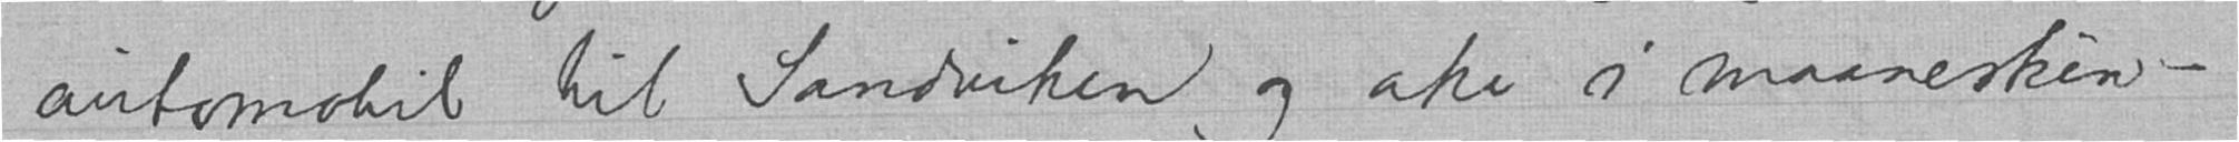antomobil til Sandviken og ake i maaneskin-
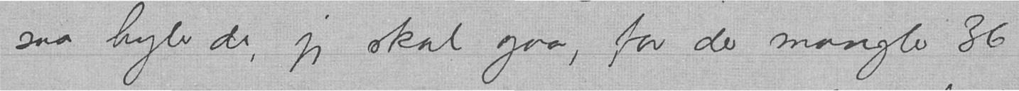saa hyler de, jeg skal gaa, for der mangler 36
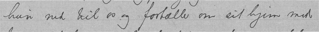han ned til os og fortæller om sit hjem med
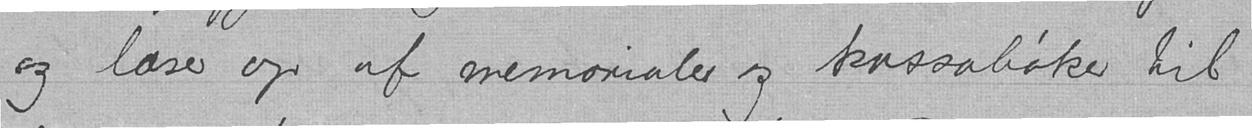og læser op af memorialer og kassabøker til
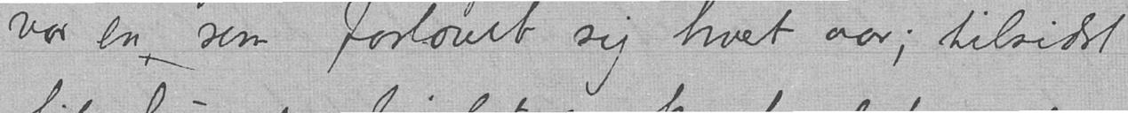var en som forlovet sig hvert aar; tilsidst
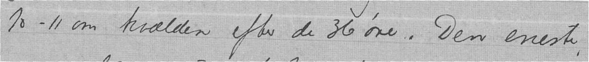10-11 om kvælden efter de 36 øre. Den eneste,
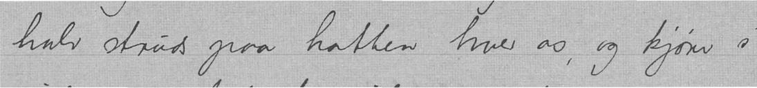halv struds paa hatten hver os, og kjøre i
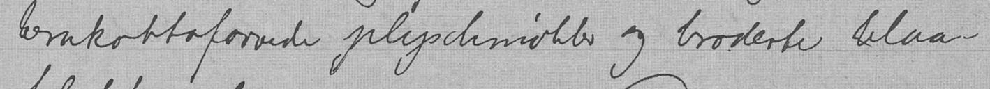terakottafarvede plyschmøbler og broderte blaa-
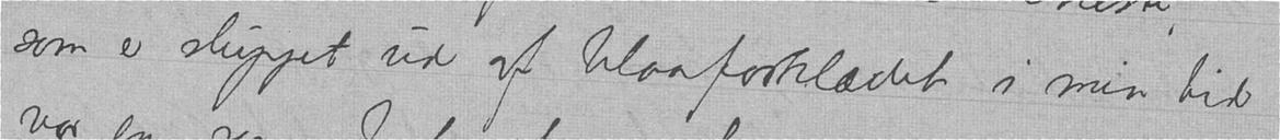som er sluppet ud af blaaforklædet i min tid
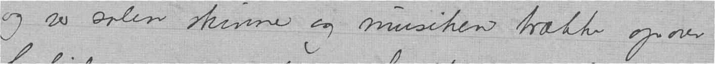og ser solen skinne og musiken trække opover
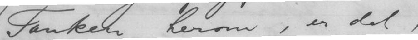Tanken herom, er det,
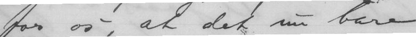for os, at det nu bare
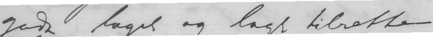godt taget og lagt tilrette
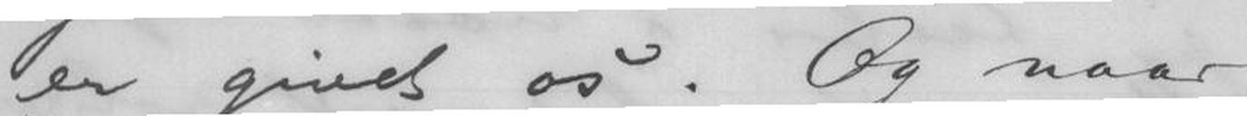er givet os. Og naar
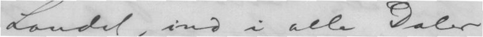Landet, ind i alle Daler,
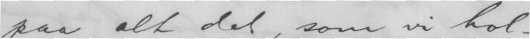paa alt det, som vi hol-
